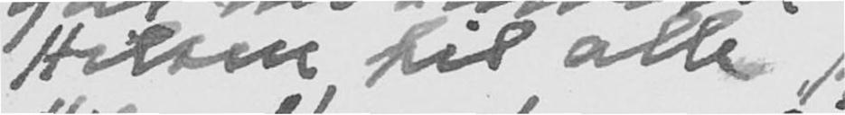Hilsen til alle
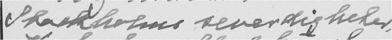Stokholms severdigheter,
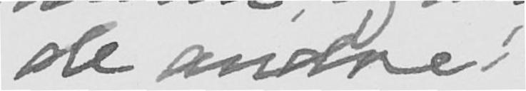de andre!
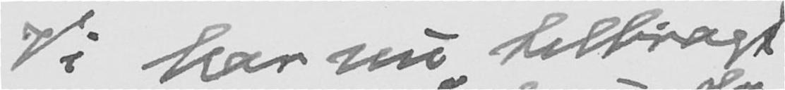Vi har nu tilbragt
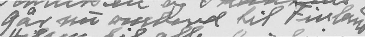Går nu ombord til Finland
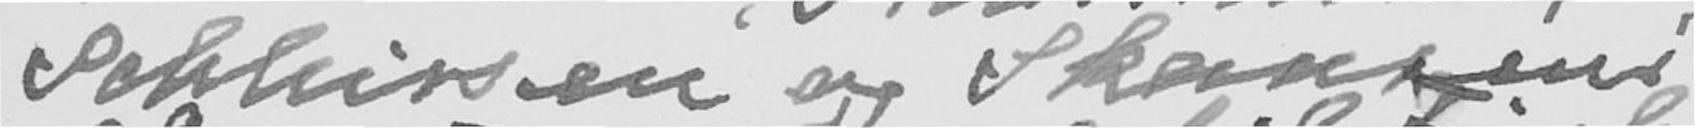Schhissen og Skansens
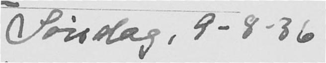Søndag, 9-8-36
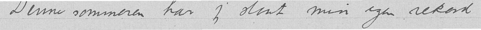Denne sommeren har jeg slaat min egen rekord
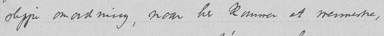slippe omordning, naar her kommer et menneske,
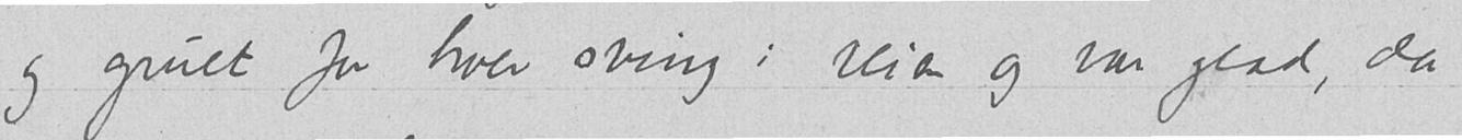og gruet for hver sving i veien og var glad, da
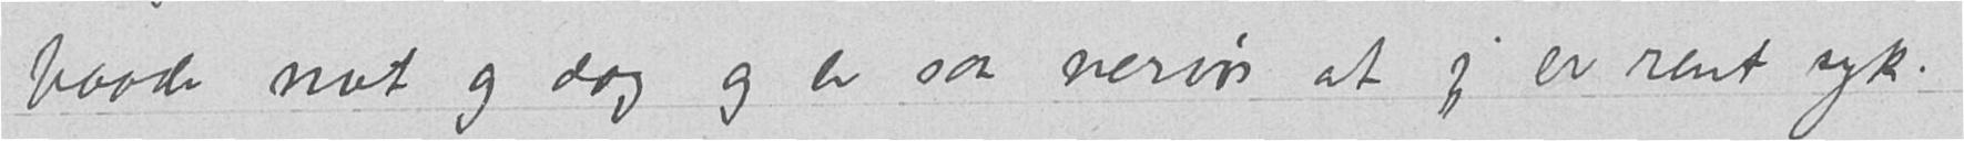baade nat og dag og er saa nervøs at jeg er rent syk.
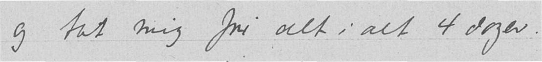og tat mig fri alt i alt 4 dager.
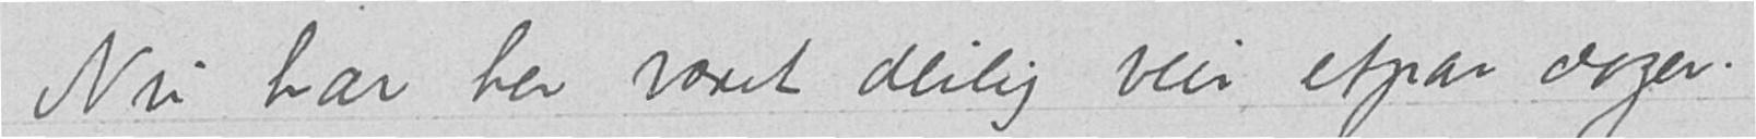Nu har her været deilig veir etpar dager.
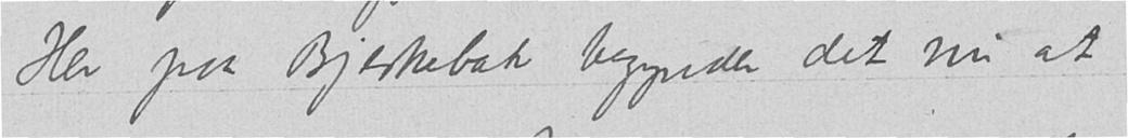Her paa Bjerkebæk begynder det nu at
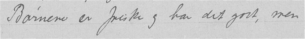Børnene er friske og har det godt, men
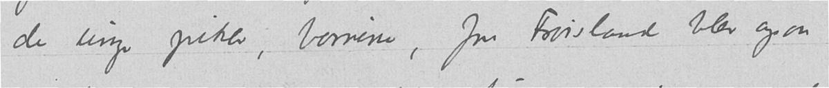de unge piker, børnene, fru Frøisland blev ogsaa
